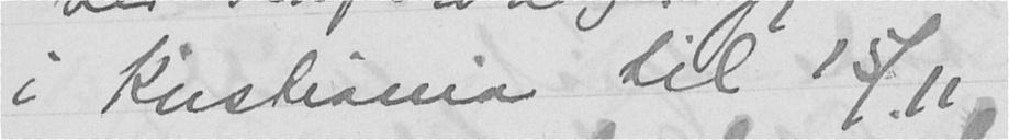i Kristiania til 15/11
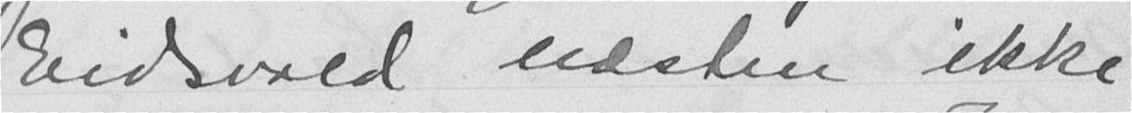Eidsvold næsten ikke
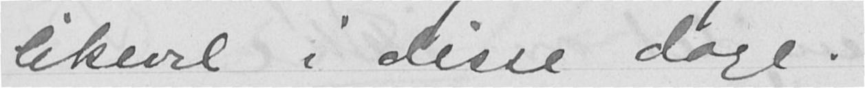likevel i disse dage.
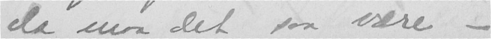da maa det saa være -
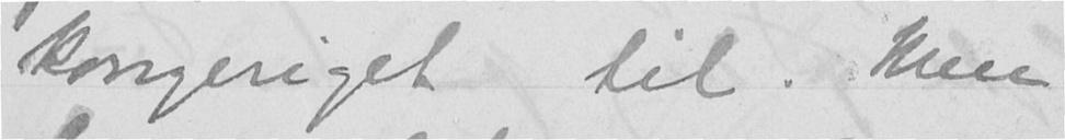kongeriget til. Men
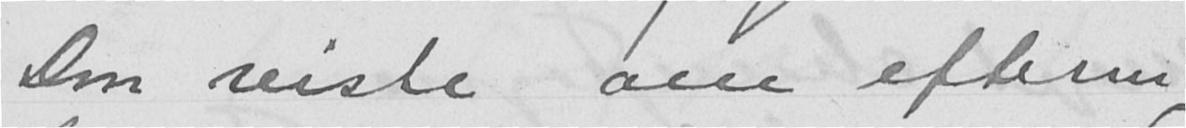Don reiste om efterm
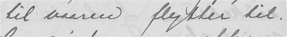til vaaren flytter til.
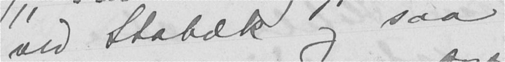ved Stabæk og saa
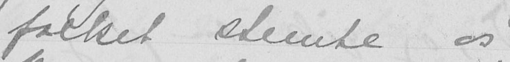folket stemte os
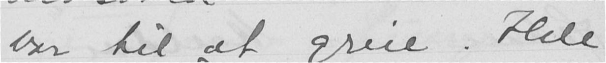var til at greie. Hele
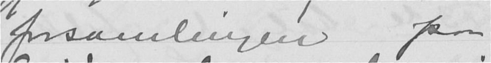forsamlingen paa
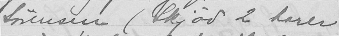Sørensen (Skjød 2 harer
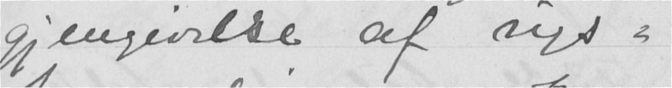gjengivelse af rigs-
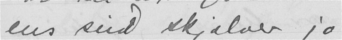ens sind skjælver jo
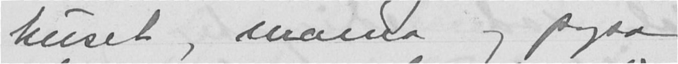huset, mama og papa
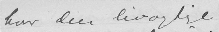hvor den livagtige
